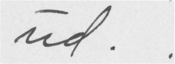ud..
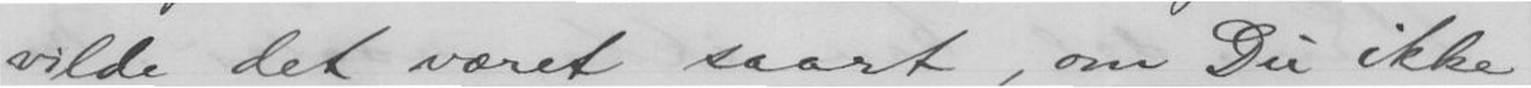vilde det været saart, om Du ikke
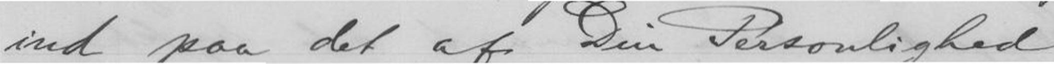ind paa det af Din Personlighed,
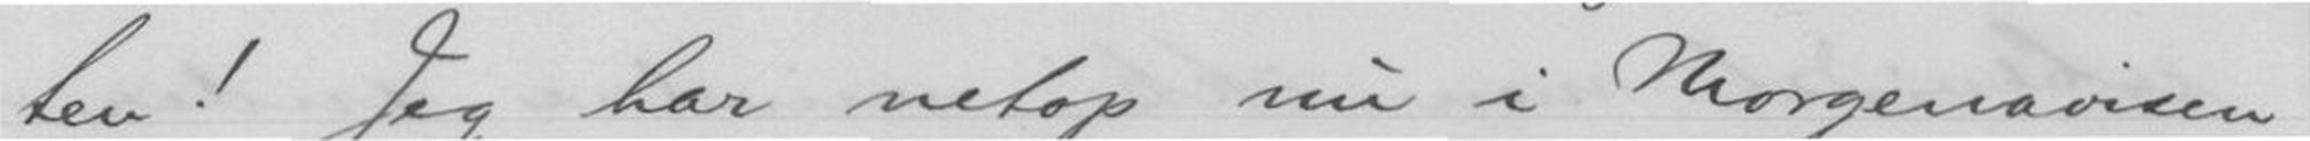ten! Jeg har netop nu i Morgenavisen
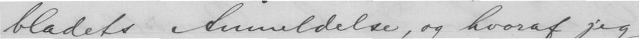bladets Anmeldelse, og hvoraf jeg
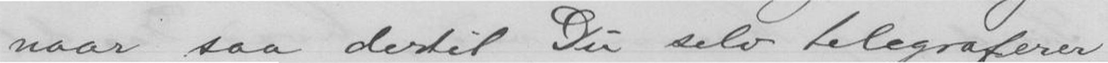naar saa dertil Du selv telegraferer
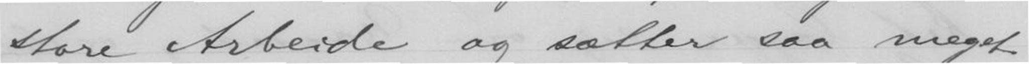store Arbeide og sætter saa meget
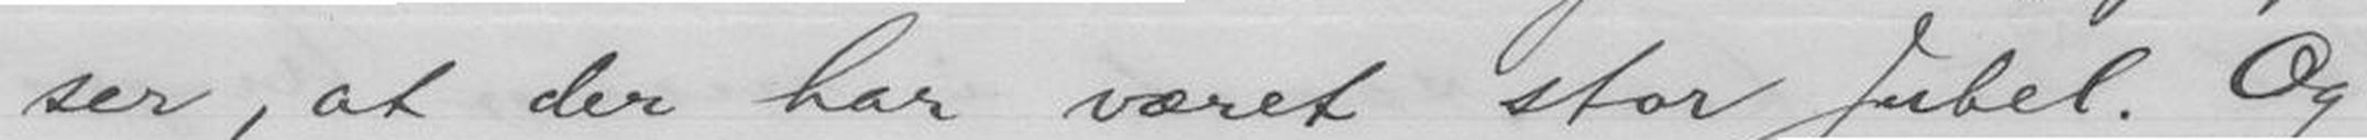ser, at der har været stor Jubel. Og
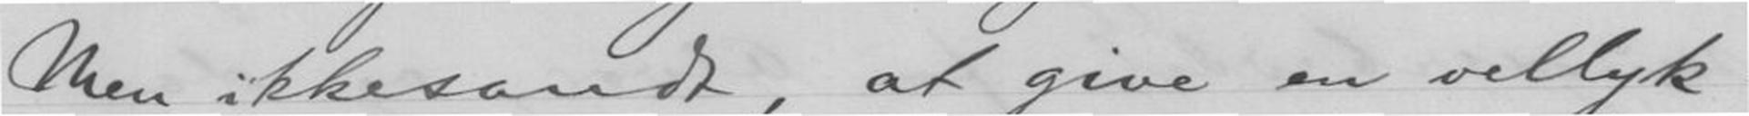Men ikkesandt, at give en vellyk-
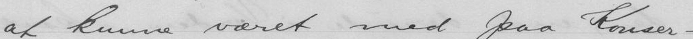at kunne været med paa Konser-
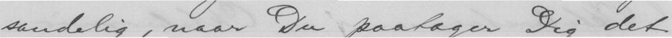sandelig, naar Du paatager Dig det
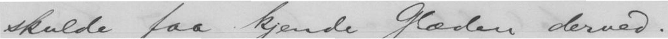skulde faa kjende Glæden derved.
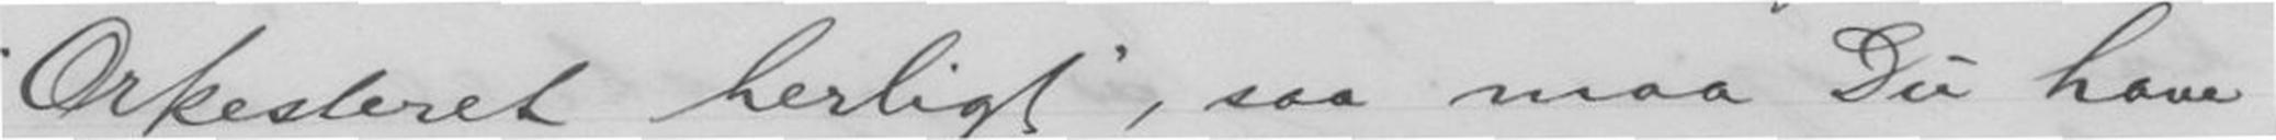"Orkesteret herligt", saa maa Du have
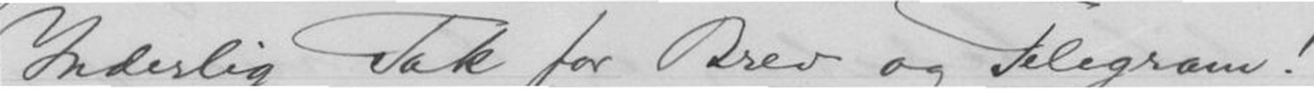Inderlig Tak for Brev og Telegram!
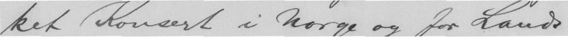ket Konsert i Norge og for Lands-
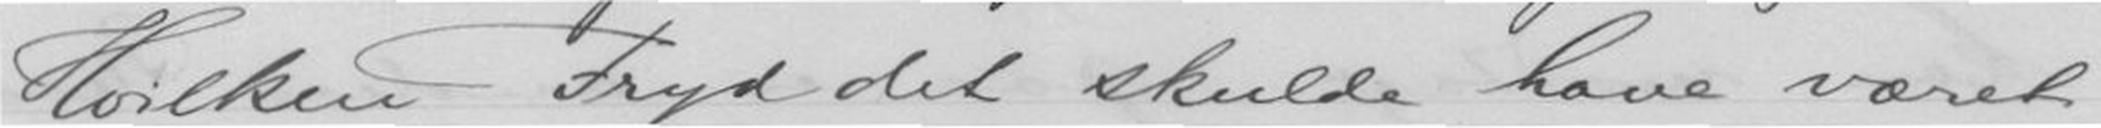Hvilken Fryd det skulde have været
